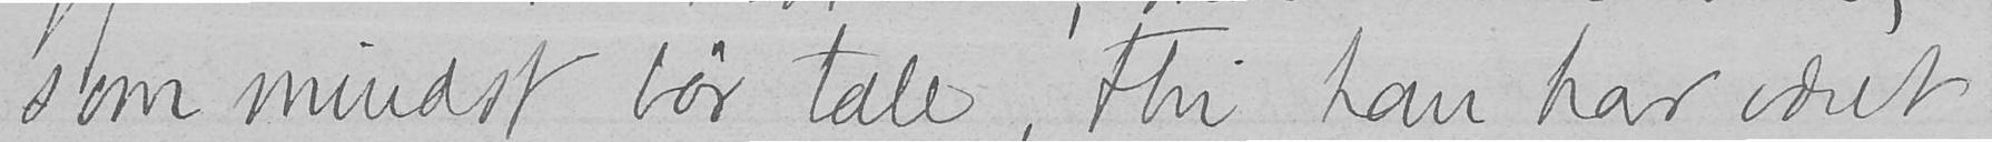som mindst bør tale, thi han har været
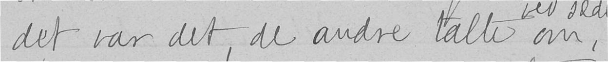det var det, de andre talte om,
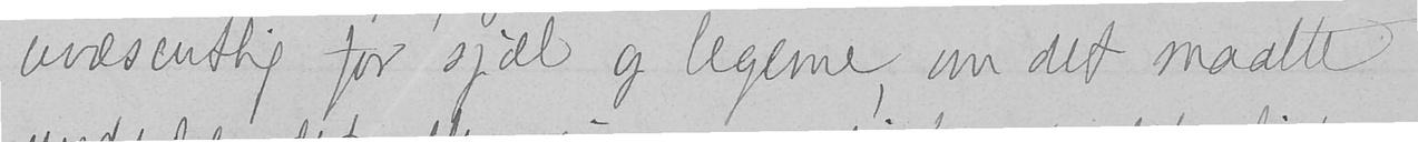uvæsentlig for sjæl og legeme, om det maatte
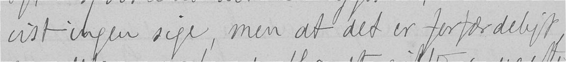vist ingen sige, men at det er forfærdeligt,
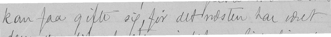kan faa gifte sig, før det næsten har været
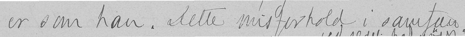er som han. Dette misforhold i samfun-
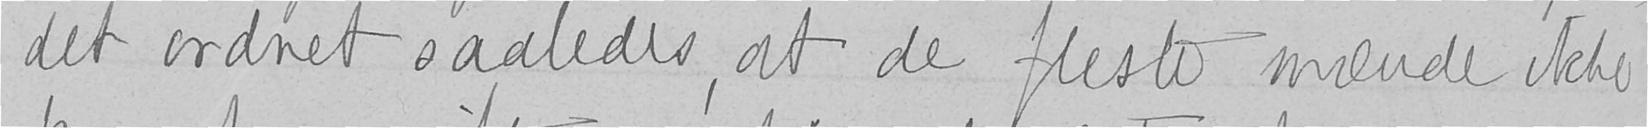det ordnet saaledes, at de fleste mænde ikke
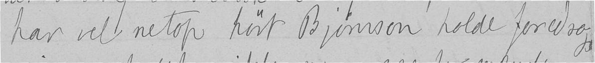har vel netop hørt Bjørnson holde foredrag
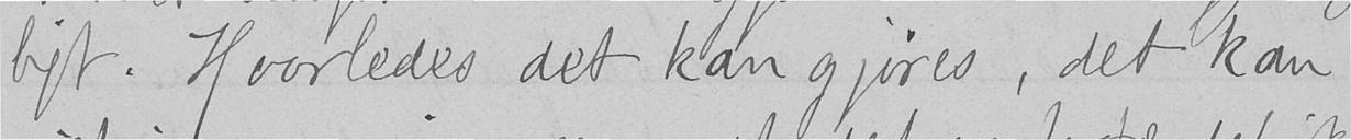ligt. Hvorledes det kan gjøres, det kan
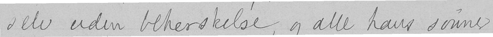selv uden beherskelse, og alle hans sønner
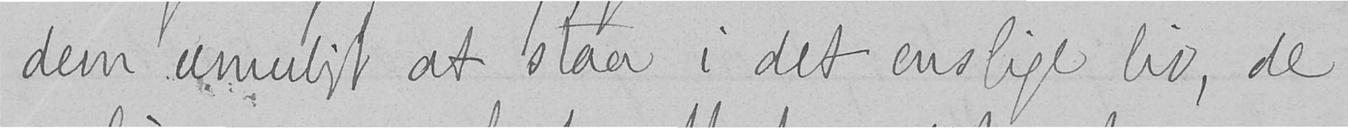dem umuligt at staa i det enslige liv, de
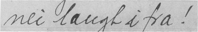nei langt i fra!
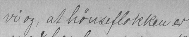vi og, at hønseflokken er
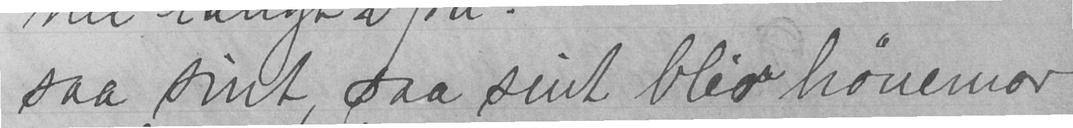saa sint, saa sint blev hønemor
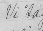Vi "ta'
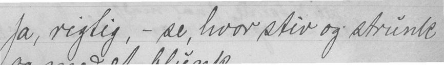Ja, rigtig, - se, hvor stiv og strunk
